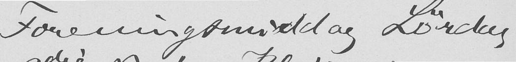Foreningsmiddag Lørdag
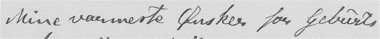Mine varmeste Ønsker for Geburts-
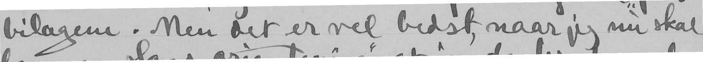bilagene. Men det er vel bedst naar jeg nu skal
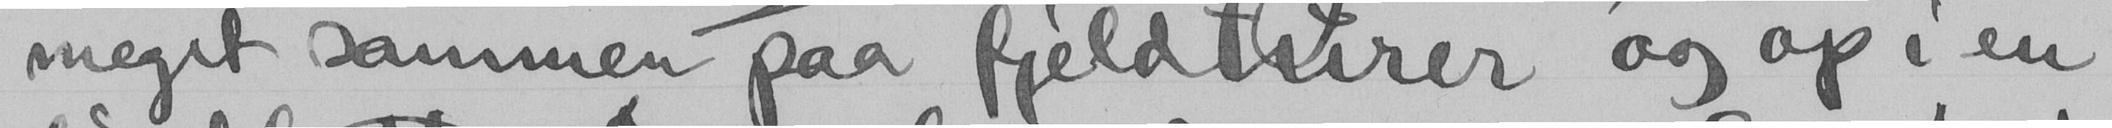meget sammen paa fjeldturer og op i en
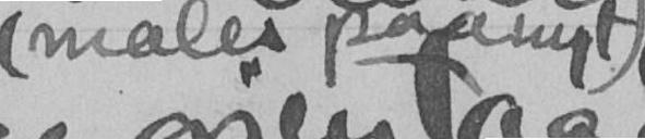(males paanyt)
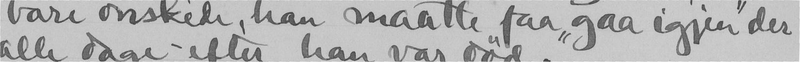bare ønskede, han maatte faa "gaa igjen" der
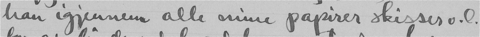han igjennem alle mine papirer skisser o.l.
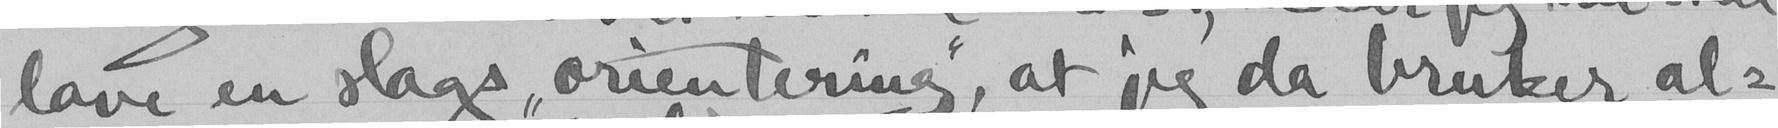lave en slags "orientering", at jeg da bruker al-
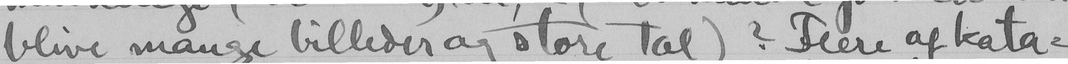blive mange billeder og store tal) ? Flere af kata-
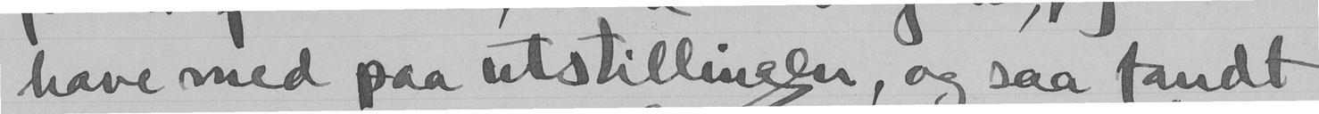have med paa utstillingen, og saa fandt
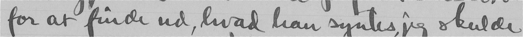for at finde ud, hvad han syntes jeg skulde
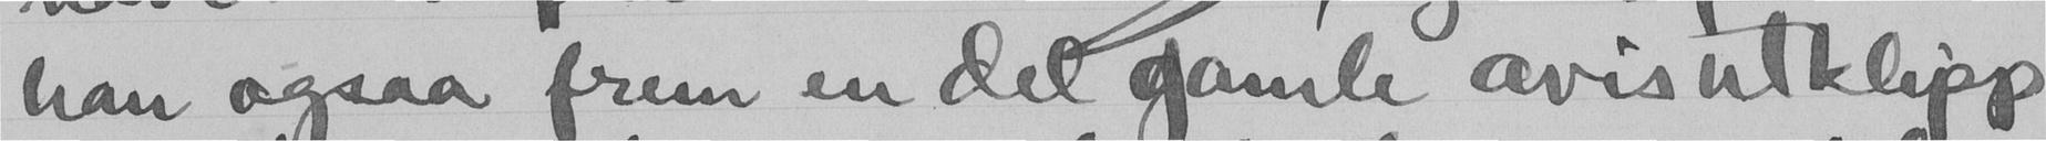han ogsaa frem en del gamle aves utklipp
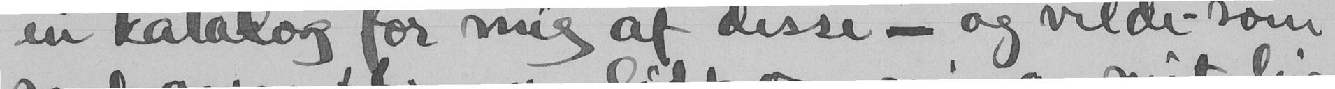en katalog for mig af disse - og vilde- som
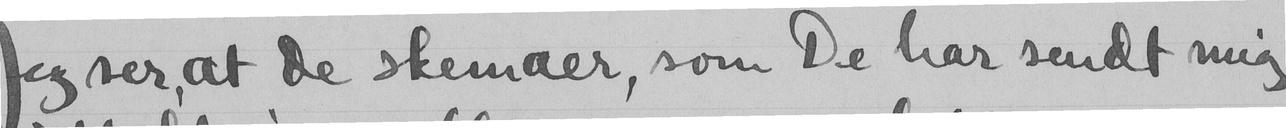Jeg ser, at de skemaer, som De har sendt mig
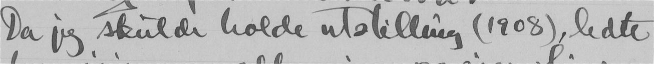Da jeg skulde holde utstilling (1908), ledte
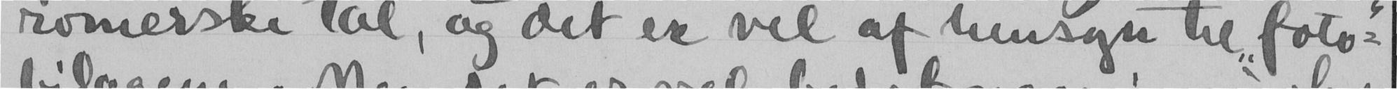romerske tal, og det er vel af hensyn til" foto"-
